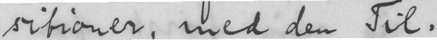sitioner, med den Til-
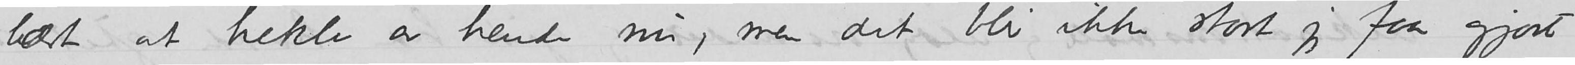lært at hekle av hende nu, men det blir ikke stort jeg faar gjort.
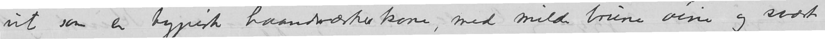ut som en typisk haandværkerkone, med milde brune øine og svært
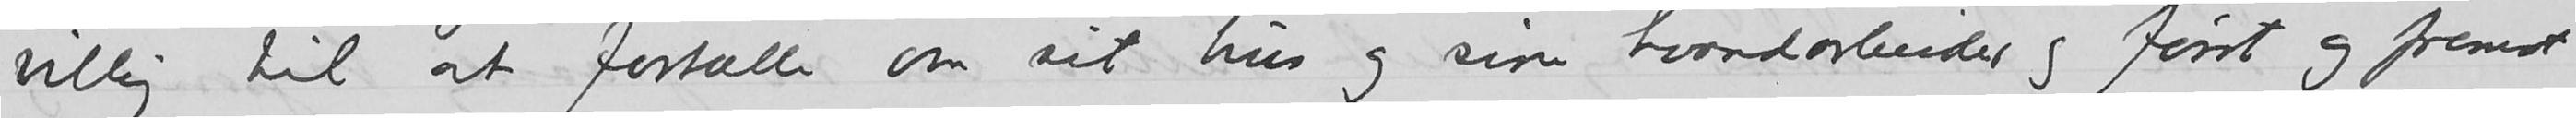villig til at fortælle om sit hus og sine haandarbeider og først og fremst
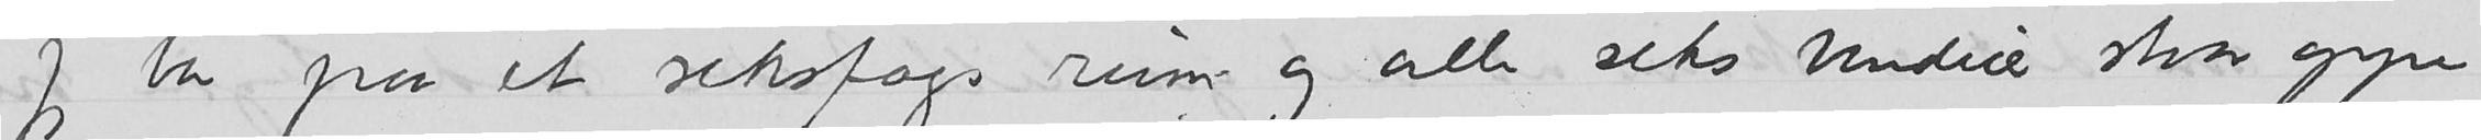Jeg bor paa et seksfags rum og alle seks vinduer staar oppe
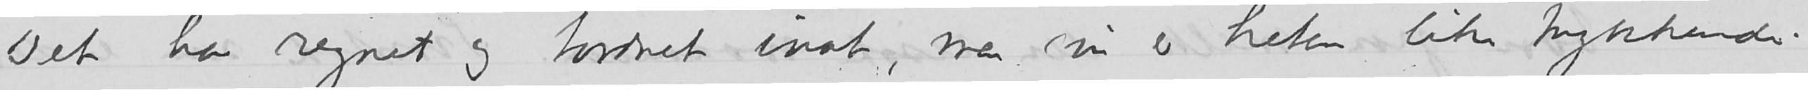Det har regnet og tordnet inat, men nu er heten like trykkende.
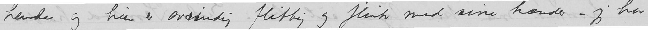hende og hun er avsindig flittig og flink med sine hænder – jeg har
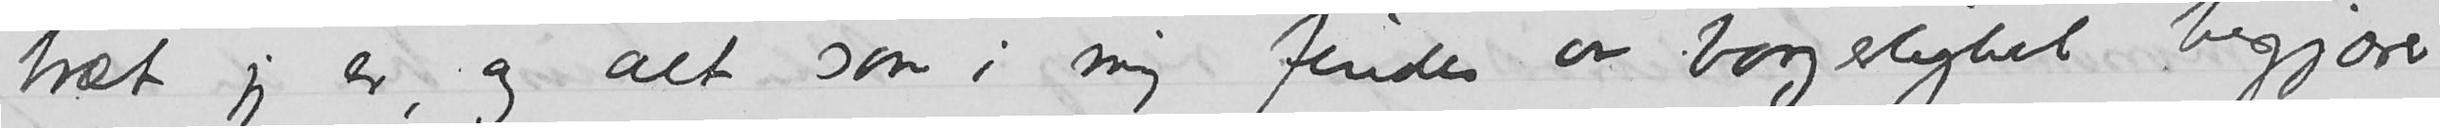træt jeg er, og alt som i mig findes av borgelighet begjærer
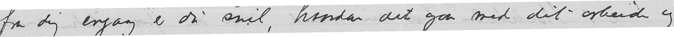fra dig engang er du snil, hvordan det gaar med dit arbeide og
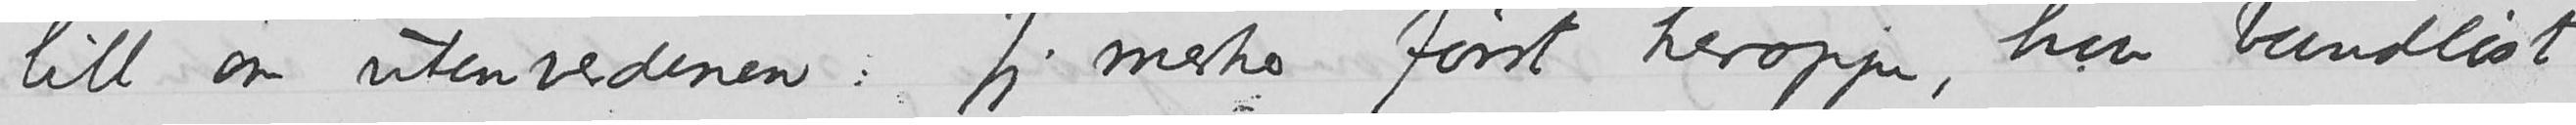litt om utenverdenen. Jeg merker først heroppe, hvor bundløst
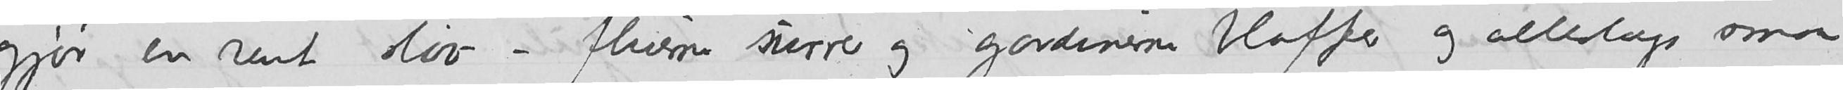gjør en rent sløv – fluerne surrer og gardinerne blaffer og alleslags smaa
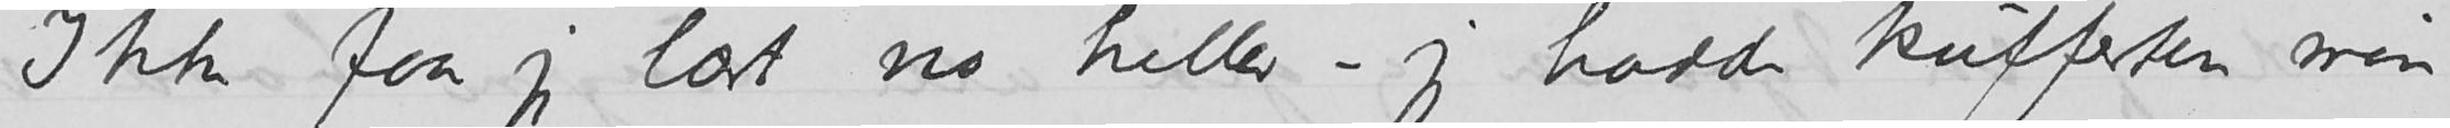Ikke faar jeg læst no heller – jeg hadde kufferten min
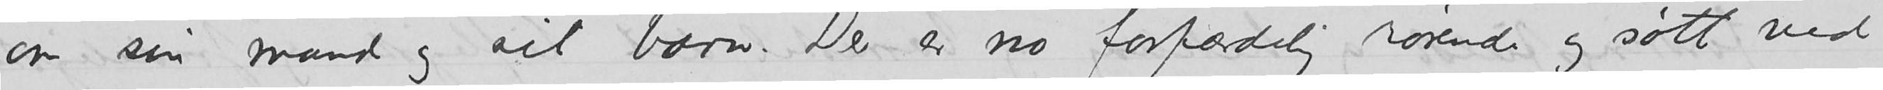om sin mand og sit barn. Det er no forfærdelig rørende og søtt ved
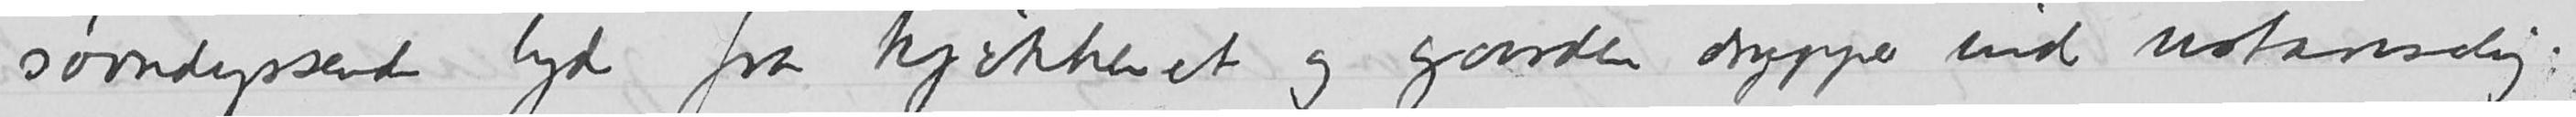søvndyssende lyd fra kjøkkenet og gaarden drypper ind ustanselig.
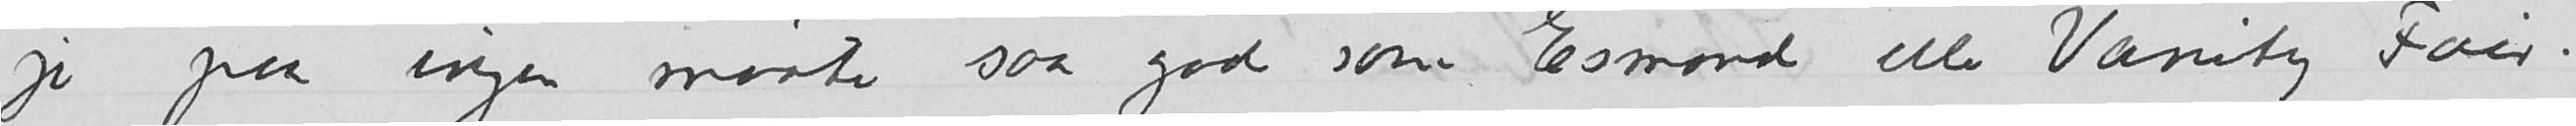jo paa ingen maate saa god som Esmond eller Vanity Fair.
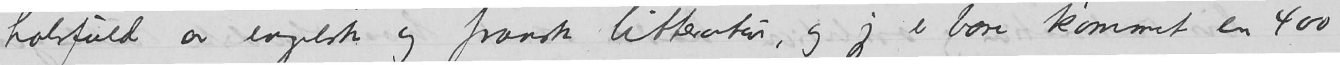halvfuld av engelsk og fransk litteratur, og jeg er bare kommet en 400
